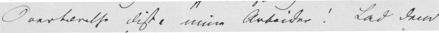Overbærelse disse mine Arbeider! Lad dem
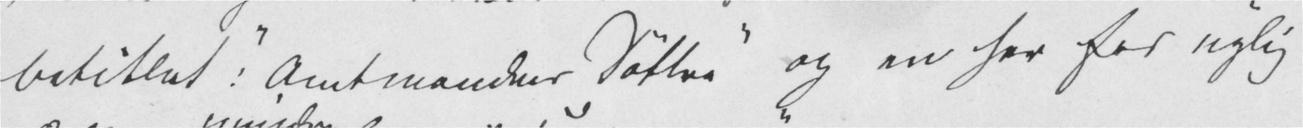betitlet: «Amtmandens Døttre» og en her for nylig
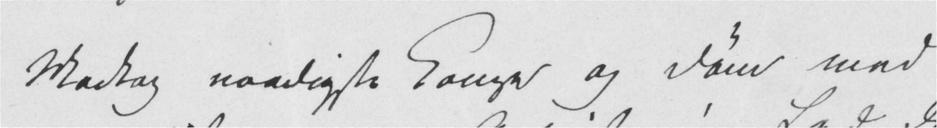Modtag naadigste Konge og døm med
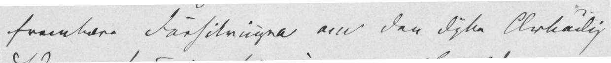frembære Forsikringen om den dybe Ærbødighed
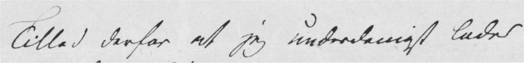Tillad derfor at jeg underdanigst lader
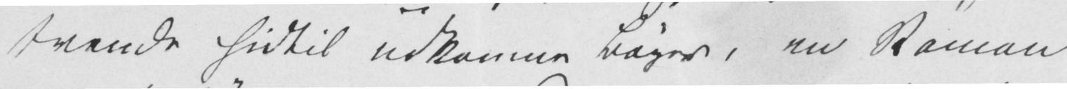tvende hidtil udkomne Bøger, en Roman
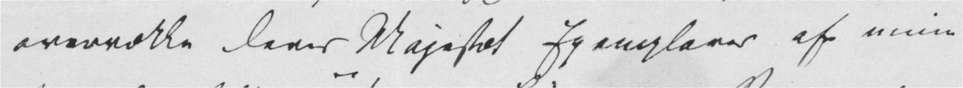overrække Deres Majestæt Exemplarer af mine
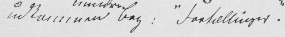udkommen Bog: «Fortællinger».
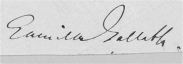Camilla Collett.
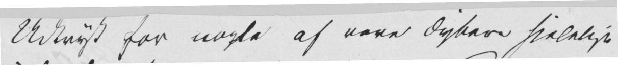Udtryk for nogle af vore dybere sjelelige
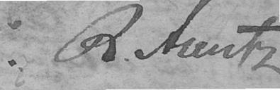A. Arentz
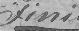Finis
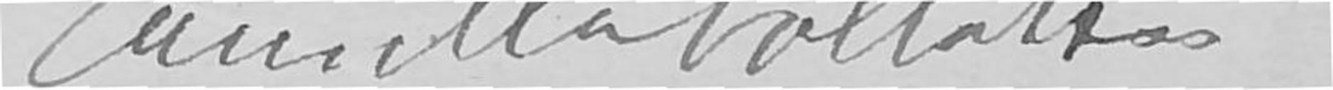Camilla Collett.
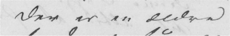Skildringer, der ogsaa have sagt at man maatte lægge Bogen bort,
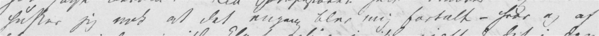husker jeg nok at det engang blev mig fortalt – hvor og af
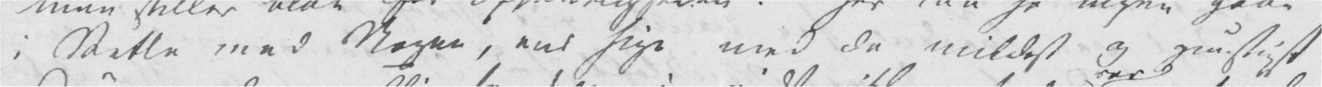i Rette med Nogen, end sige med de mildest og gunstigst
